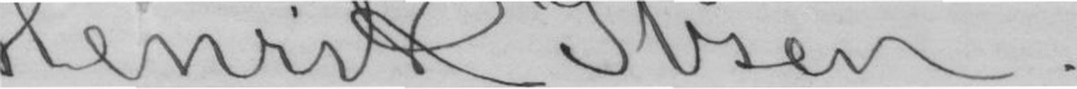Henrik Ibsen.
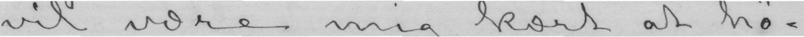vil være mig kært at hø-
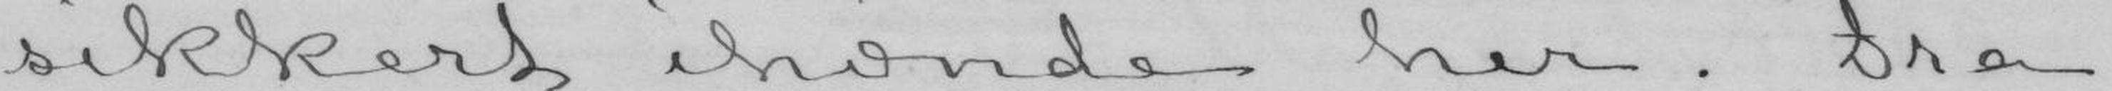sikkert ihænde her. Fra
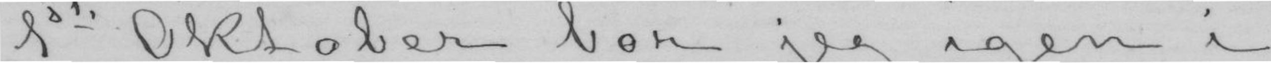1ste Oktober bor jeg igen i
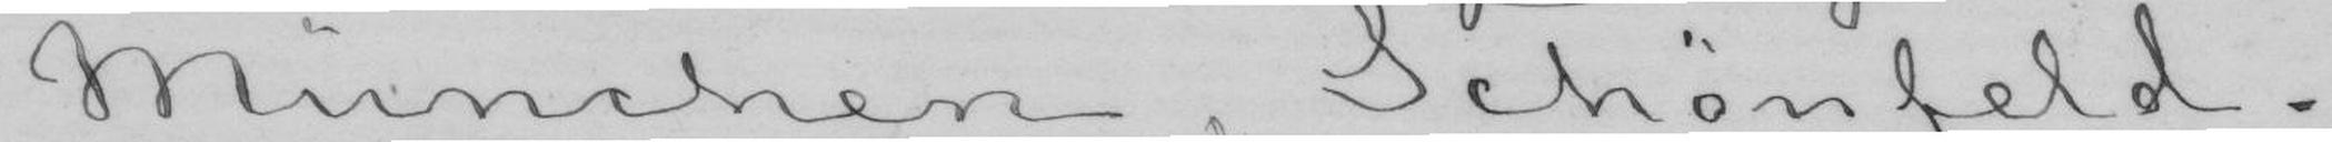München, Schönfeld-
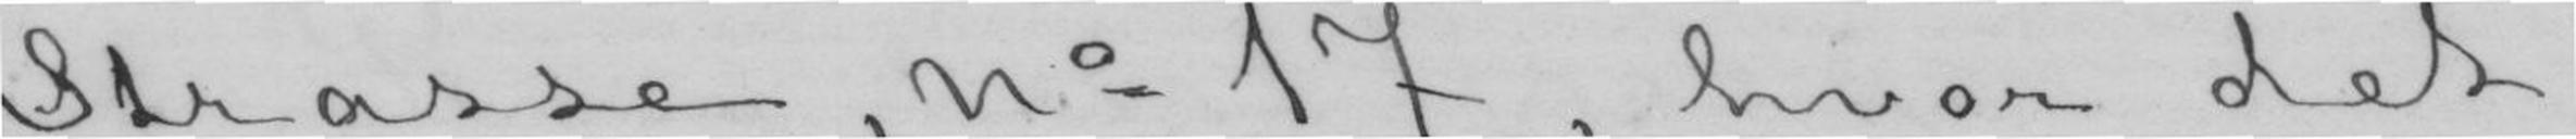strasse, N. 17, hvor det
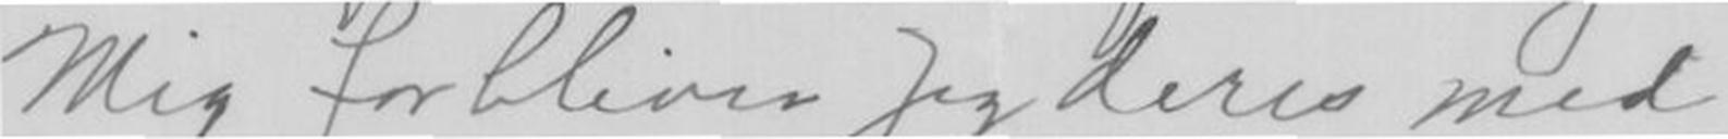Mig forbliver Jeg deres med
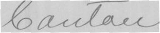Canton
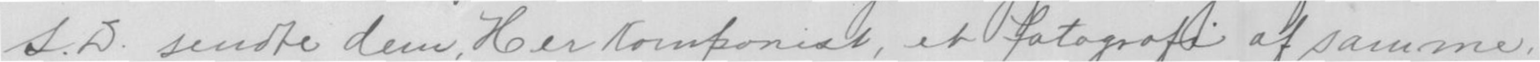S. D. sendte dem, Her Komponist, et fotografi af samme,
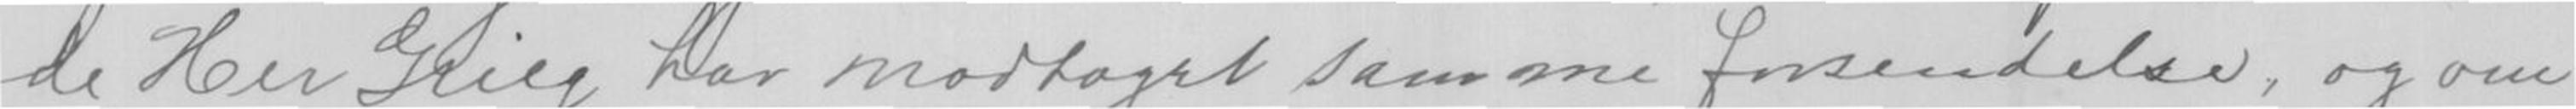de Her Grieg har modtaget samme forsendelse, og om
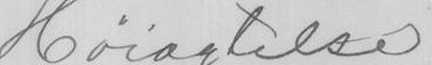Høiagtelse
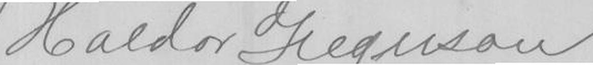Haldor Greguson
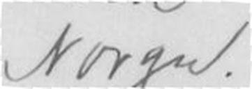Norge.
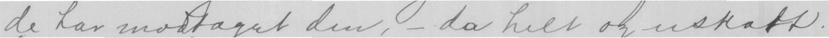de har modtaget den, - da helt og uskatt.
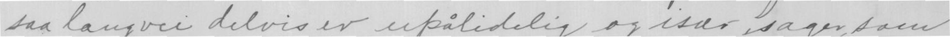saa langvei delvis er upålidelig og især sager, som
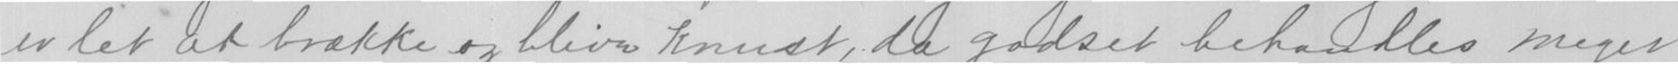er let at brække og blive knust, da godset behandles meget
